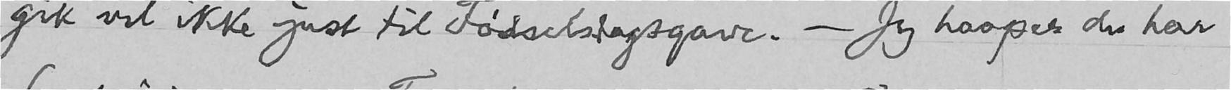gik vel ikke just til Fødselsdagsgave. - Jeg haaper du har
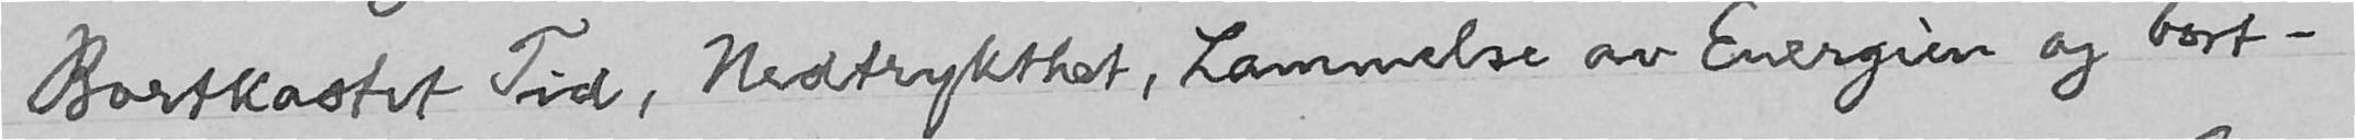Bortkastet Tid, Nedtrykthet, Lammelse av Energien og bort-
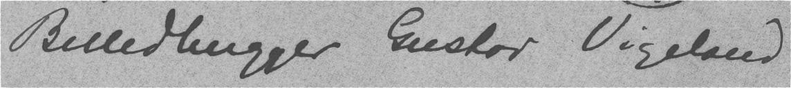Billedhugger Gustav Vigeland
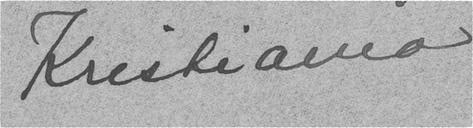Kristiania
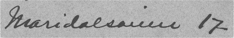Maridalsveien 17
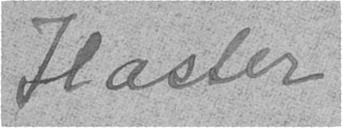Haster
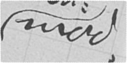mod-
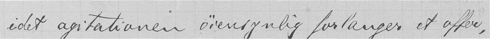idet agitationen øiensynlig forlanger et offer,
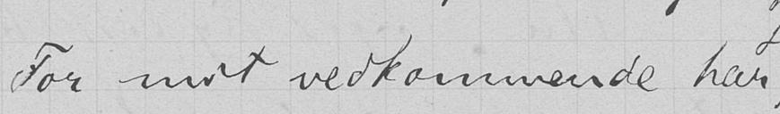For mit vedkommende har
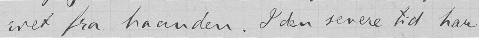riet fra haanden. I den senere tid har
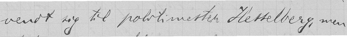vendt sig til politimester Hesselberg, men
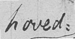hoved-
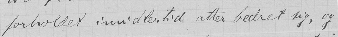forholdet imidlertid atter bedret sig, og
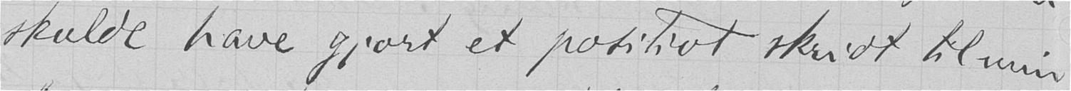skulde have gjort et positivt skridt til min
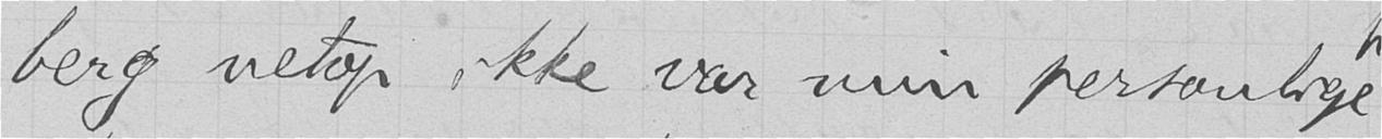berg netop ikke var min personlige
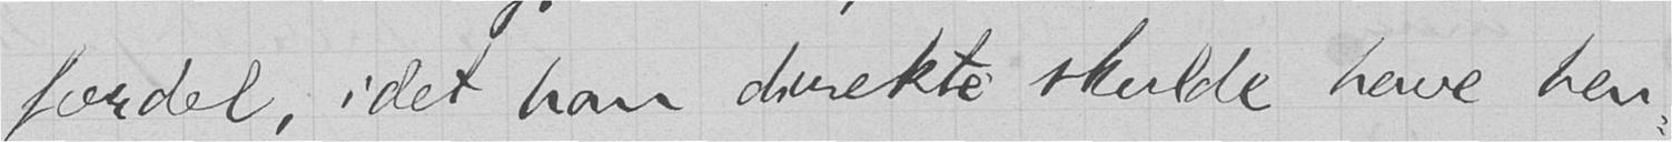fordel, idet han direkte skulde have hen-
